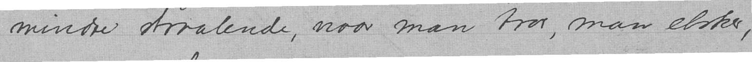mindre straalende, naar man tror, man elsker,
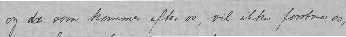og de som kommer efter os, vil ikke forstaa os,
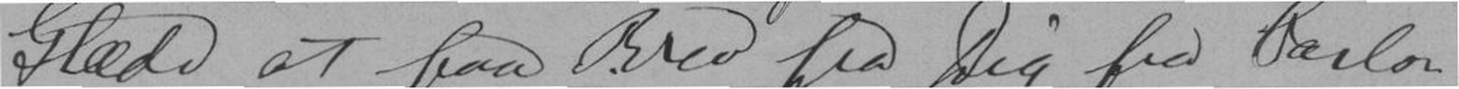Glæde at faa Brev fra Dig fra Carls-
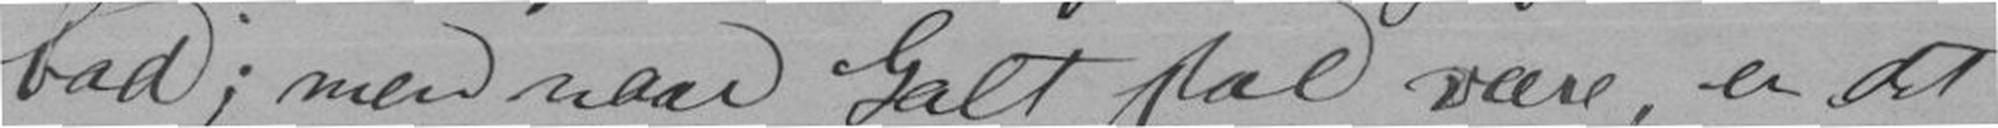bad; men naar Galt skal være, er det
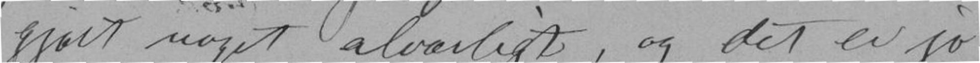gjort noget alvorligt, og det er jo
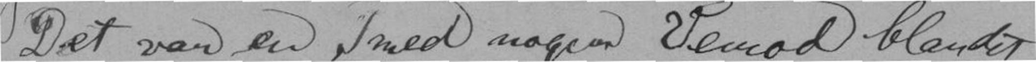Det var en I med nogen Vemod blandet
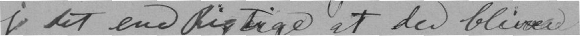jo det ene Rigtige at der bliver
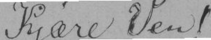Kjære Ven!
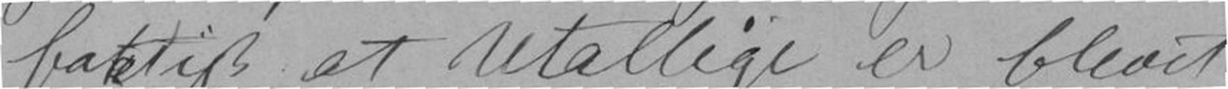faktisk, at Utallige er blevet
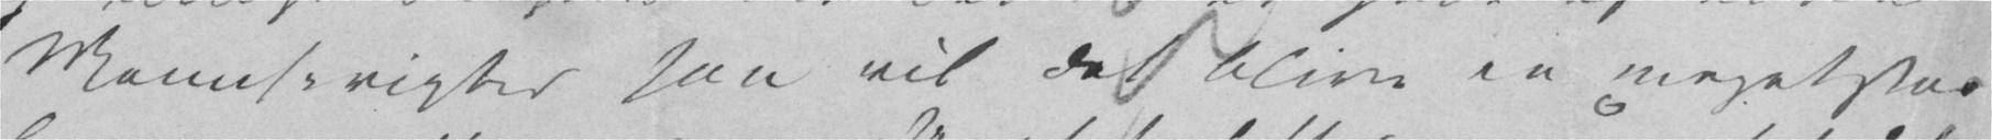Manuscripter saa vil det blive en meget stor
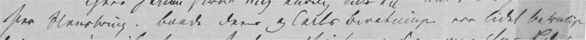fra Stenstrup. Baade Deres og Carls Beretninger ere lidet beroligen
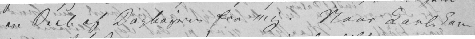en Deel af Dagbøgerne fra mig. Naar Carl kom
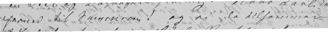herned til Sommeren! og vi da tilsammen
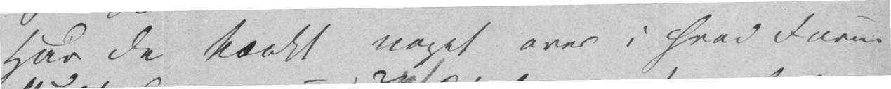Har De tænkt noget over i hvad Form
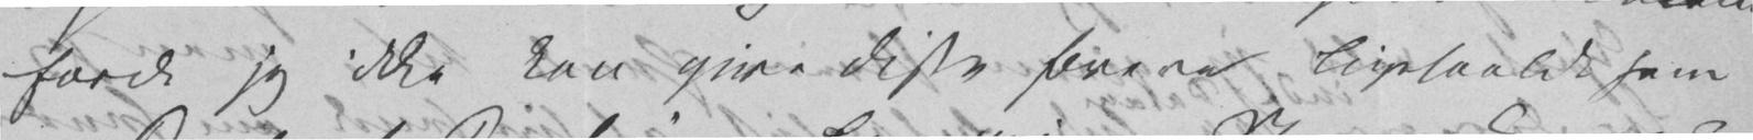fordi jeg ikke kan give disse Breve ligesaalidt som
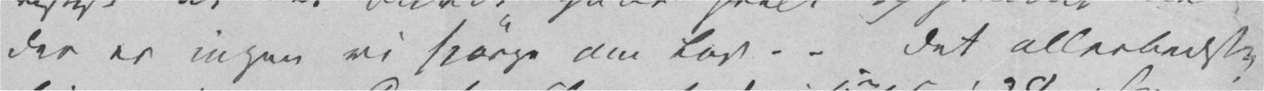der er ingen vi spørge om Lov – – Det allerbedste
